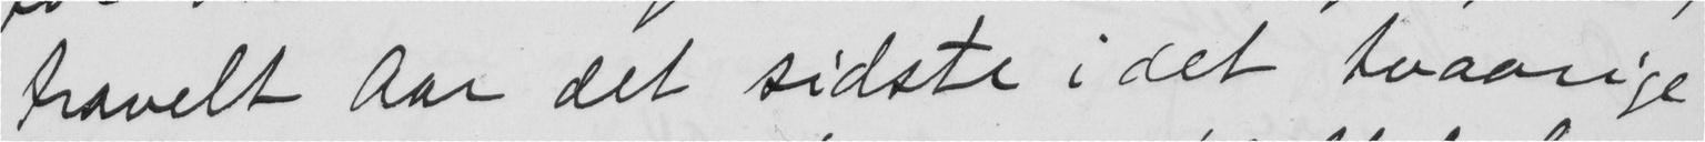travelt Aar det sidste i det tvaarige
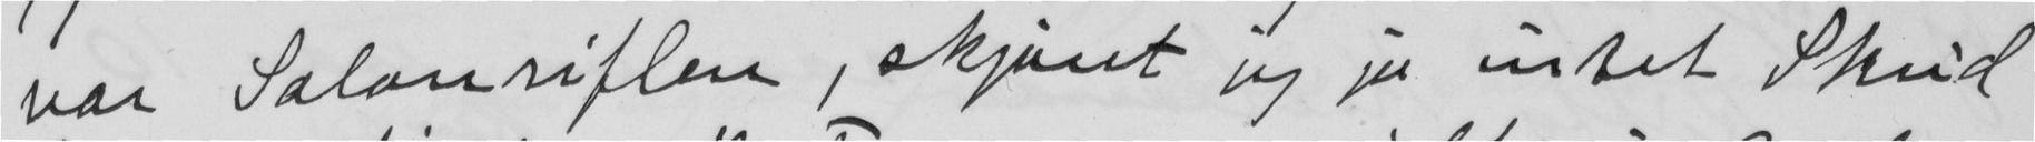var Salonriflen, skjønt jeg jo intet Skud
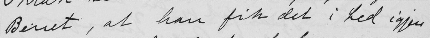Benet, at han fik det i Led igjen
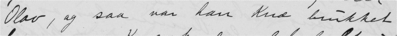Olav, og saa var han knæ brukket
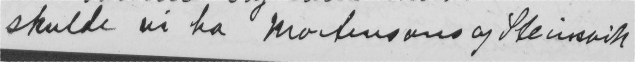skulde vi ha Mortensons og Steinsvik
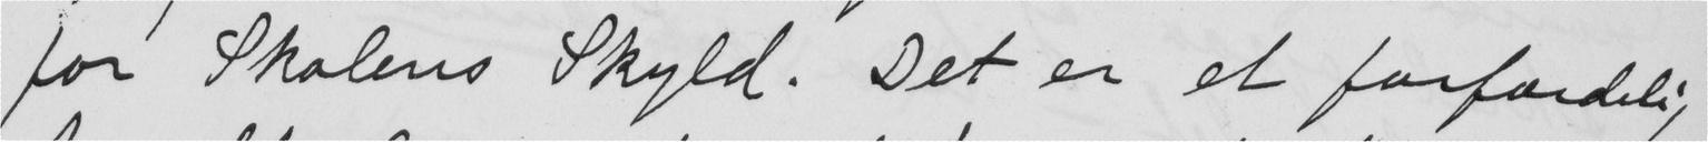for Skolens Skyld. Det er et forfærdelig
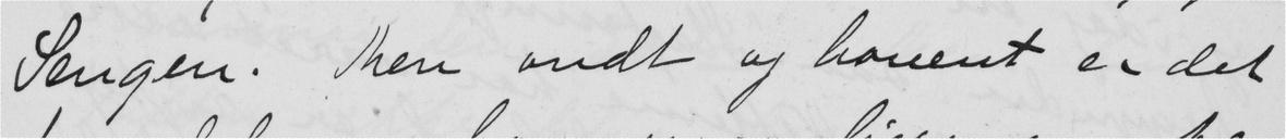Sengen. Men ondt og hovent er det
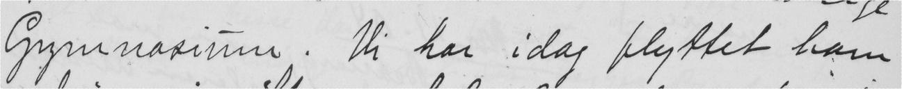Gymnasium. Vi har idag flyttet ham
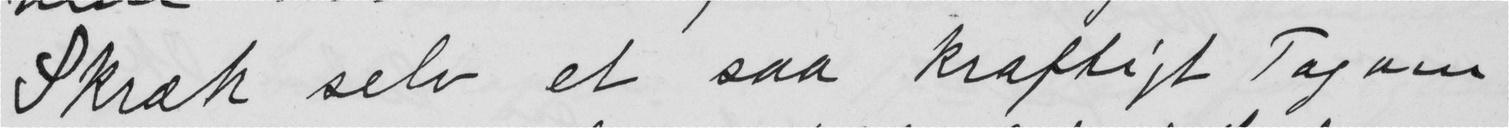Skræk selv et saa kraftigt Tag om
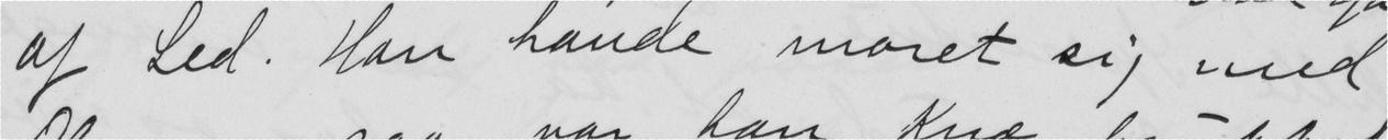af Led. Han havde moret sig med
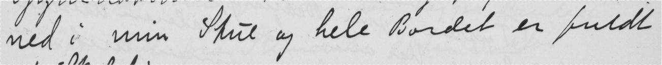ned i min Stue og hele Bordet er fuldt
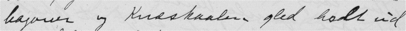bagover og Knæskaalen gled helt ud
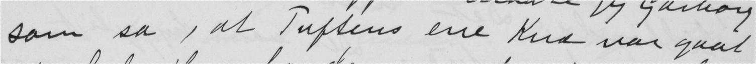som sa, at Tuftens ene Knæ var gaat
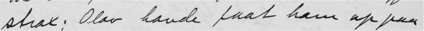strax; Olav havde faat ham op paa
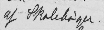af Skolebøger.
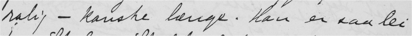rolig - kanske længe. Han er saa lei
